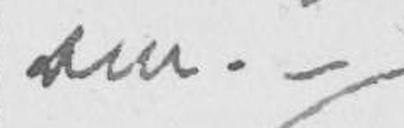om. -
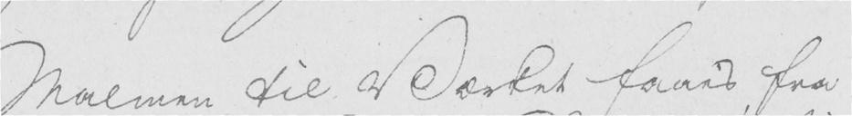Malmen til Værket faaes fra
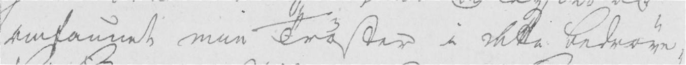omfaunet min Trøster i dette bedrøve-
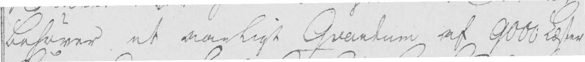behøver et aarligt Qvantum af 9000 Læster
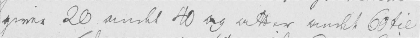giver 20 andet 40 og atter andet 60 til
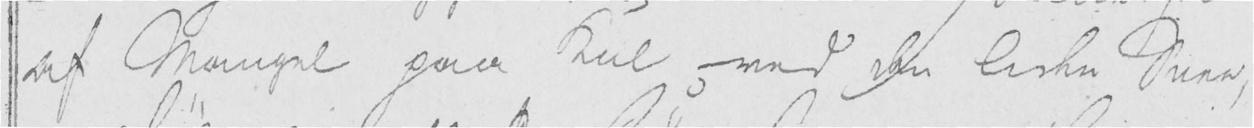af Mangel paa Kul ved den liden Snee,
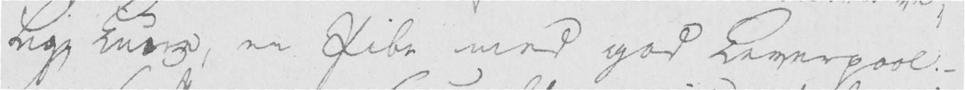lige Huus, en Pibe med god Liverpool. -
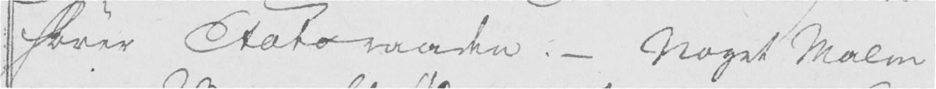hører Etatsraaden. - Noget Malm
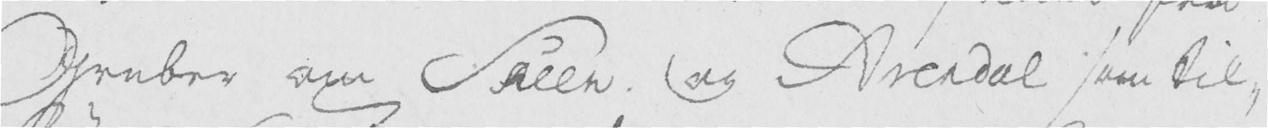Gruber om Skeen og Arendal som til-
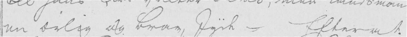en ærlig og brav, Jyde - Efterat
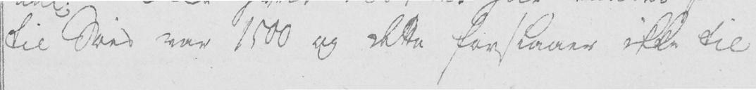til Sais var 1500 og dette forsbaaer ikke til
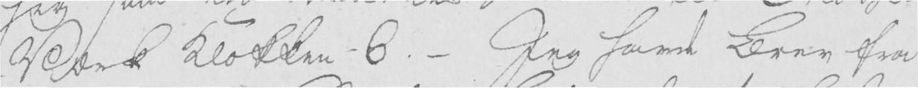Værk Klokken 6. - Jeg havde Brev fra
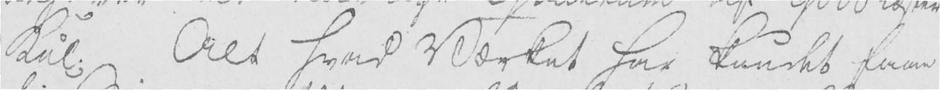Kul; Alt hvad Værket har kundet faae
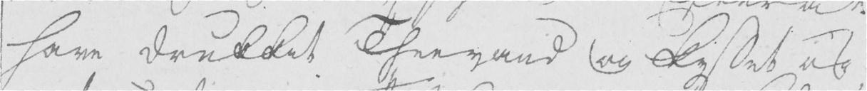have drukket Theevand og kysset og
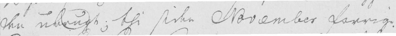den ubrugt; thi siden November forrige
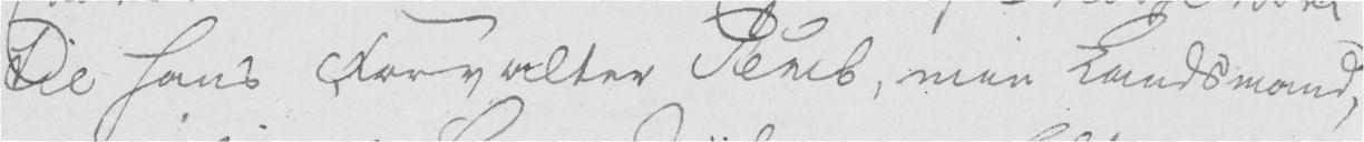til hans Forvalter Semb, min Landsmand,
